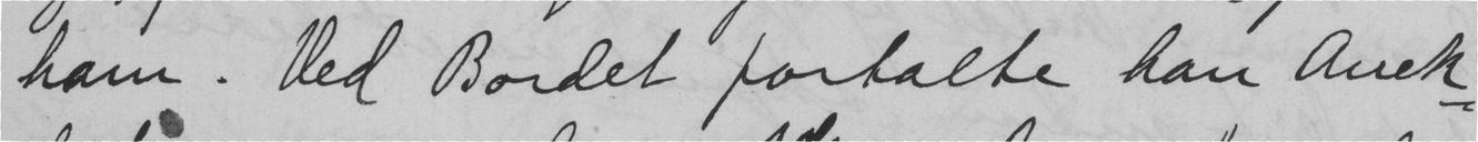ham. Ved Bordet fortalte han anek-
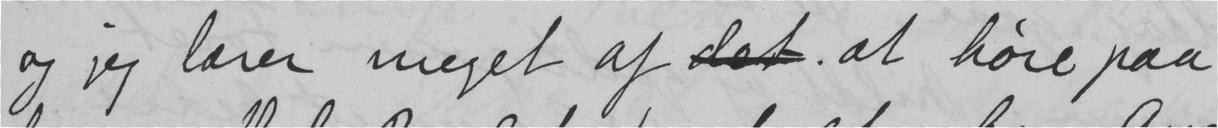og jeg lærer meget af det, at høre paa
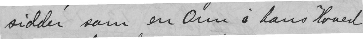sidder som en ovn i hans Hoved
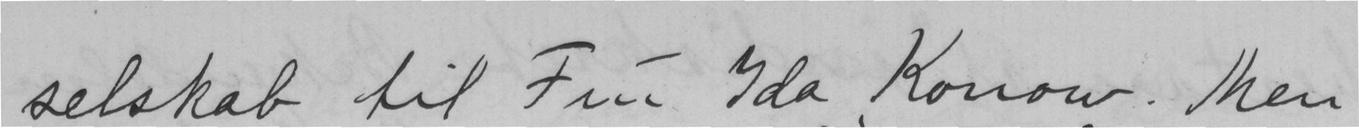selskab til Fru Ida Konow. Men
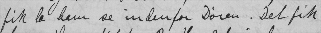fik la ham se indenfor Døren. Det fik
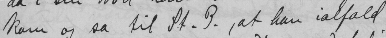kom og sa til St. P., at han ialfald
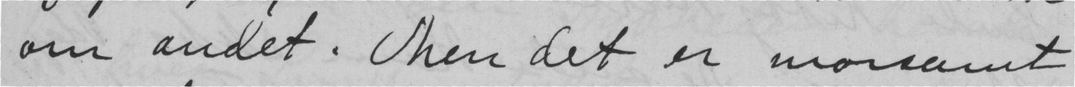om andet. Men det er morsomt
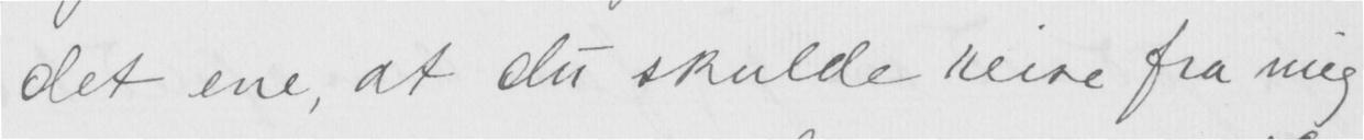det ene, at du skulde reise fra mig
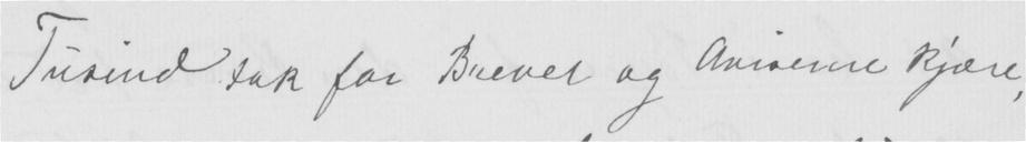Tusind tak for Brever og Aviserne kjære,
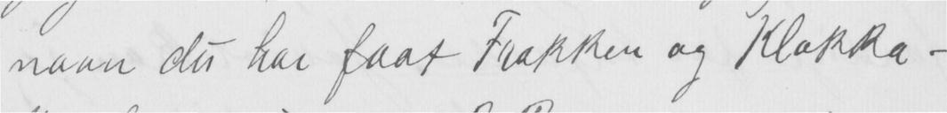navn du har faat Frakken og Klokka –
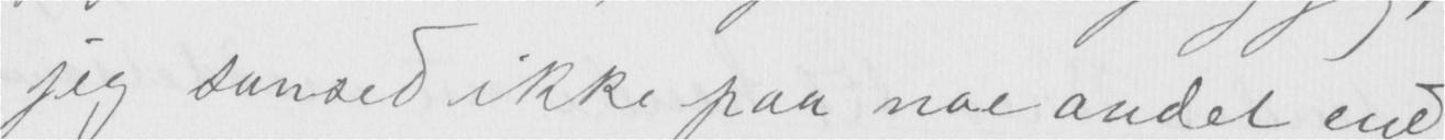jeg sansed ikke paa noe andet end
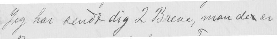Jeg har sendt dig 2 Breve, mon der er
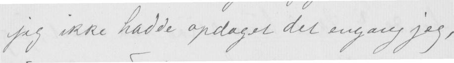jeg ikke hadde opdaget det engang jeg,
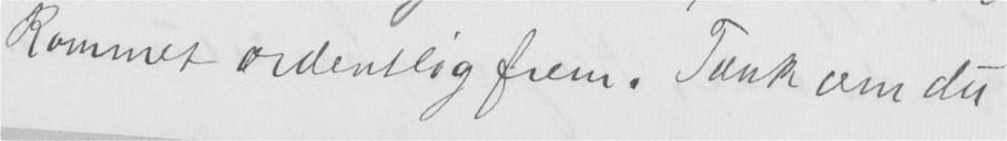kommet ordentlig frem. Tænk om du
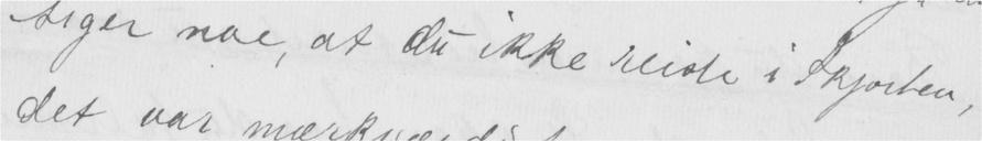siger noe, at du ikke reiste i Skjorten,
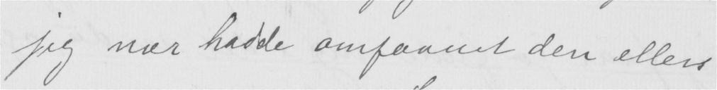jeg nær hadde omfavnet den ellers
